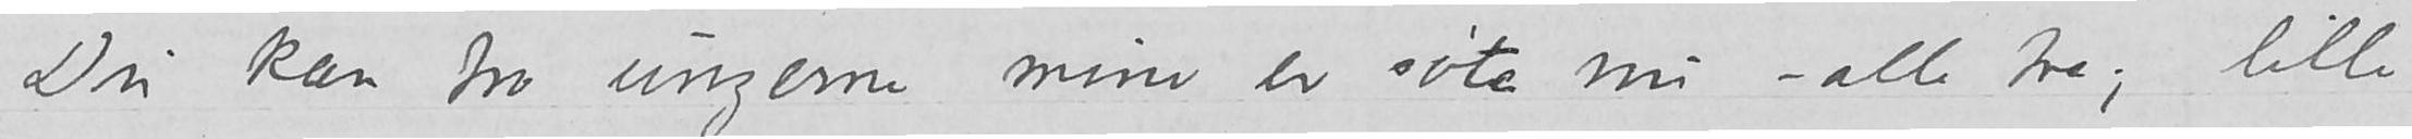Du kan tro ungerne mine er søte nu – alle tre; lille
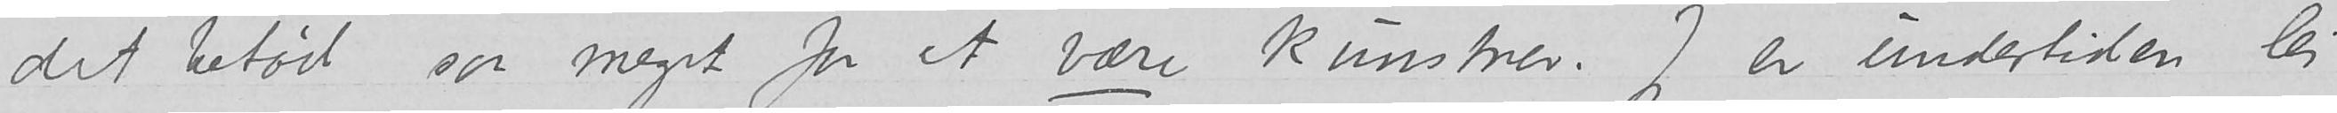det betød saa meget for at være kunstner. Jeg er undertiden lei
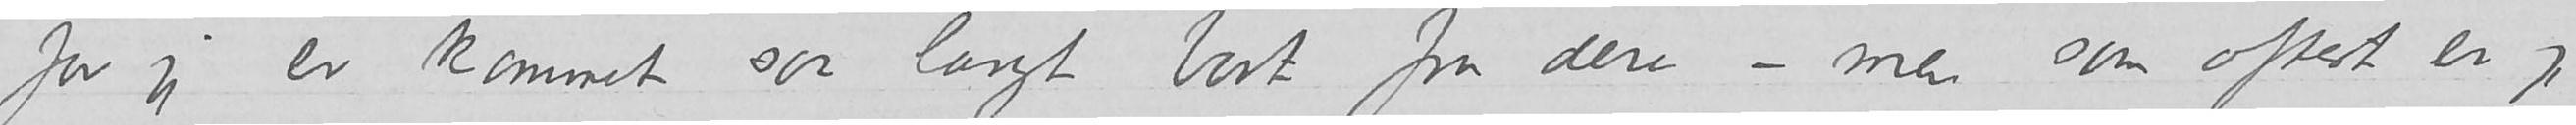for jeg er kommet saa langt bort fra dere – men som oftest er jeg
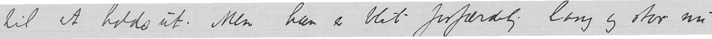til at holde ut. Men han er blit forfærdelig lang og stor nu
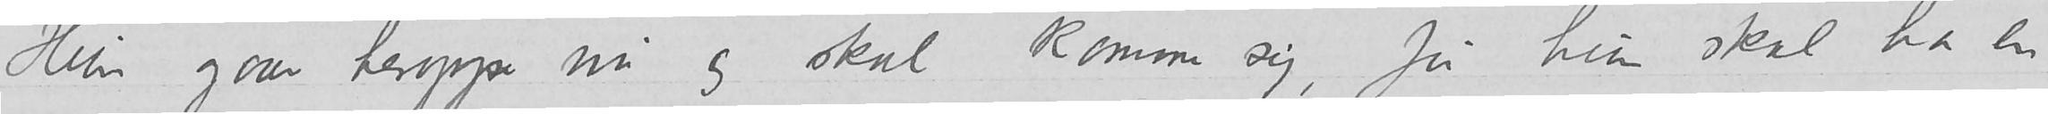Hun gaar heroppe nu og skal komme sig, før hun skal ha en
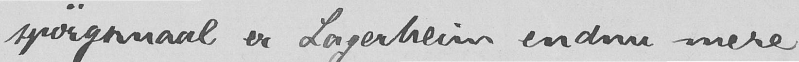spørgsmaal er Lagerheim endnu mere
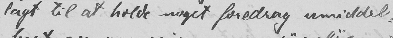lagt til at holde noget foredrag umiddel-
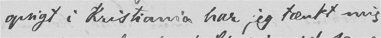opsigt i Kristiania har jeg tænkt mig,
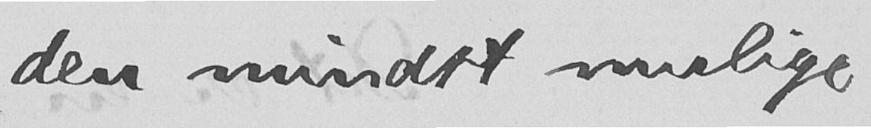den mindst mulige
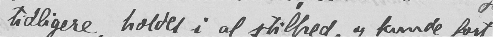tidligere, holdes i al stilhed, og kunde fort-
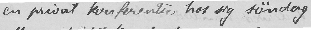en privat konferentse hos sig søndag
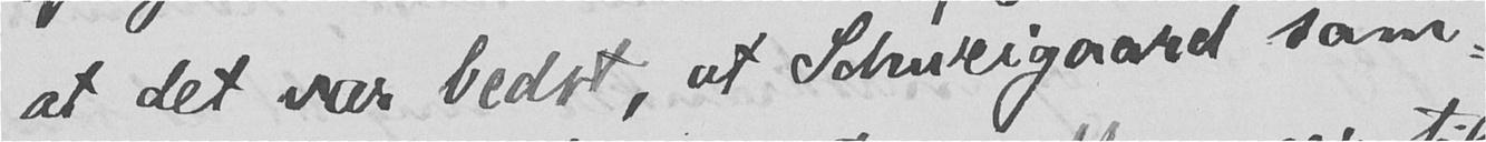at det var bedst, at Schweigaard sam-
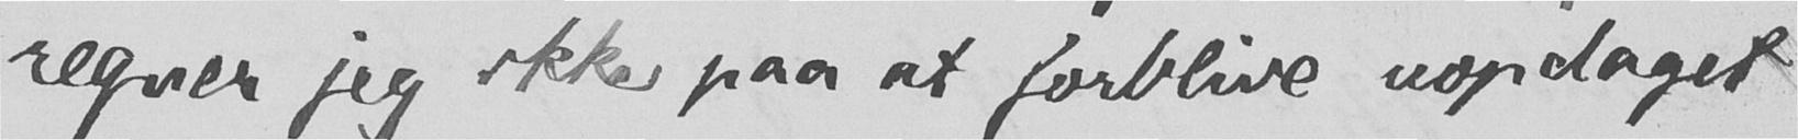regner jeg ikke paa at forblive uopdaget
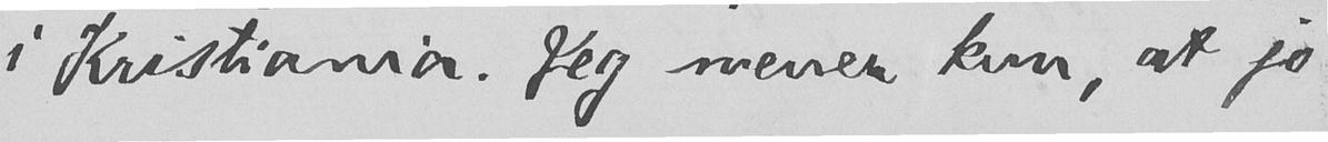i Kristiania. Jeg mener kun, at jo
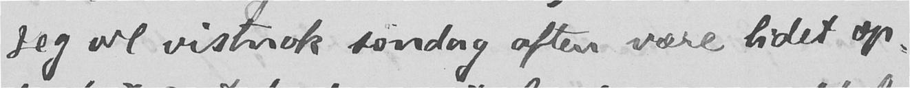Jeg vil vistnok søndag aften være lidet op-
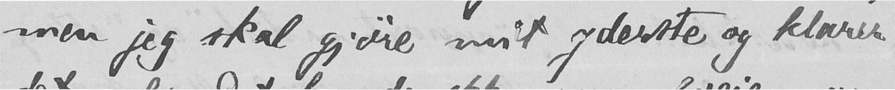men jeg skal gjøre mit yderste og klarer
